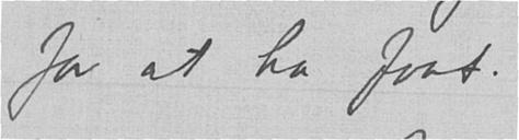for at ha faat.
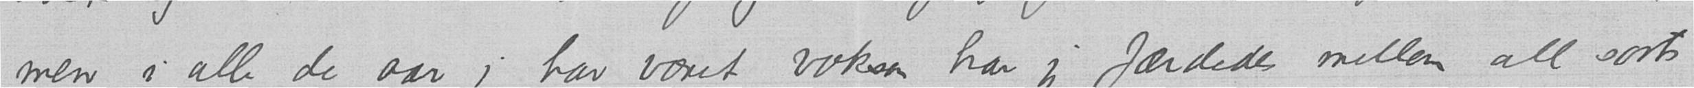men i alle de aar jeg har været voksen har jeg færdedes mellem all sorts
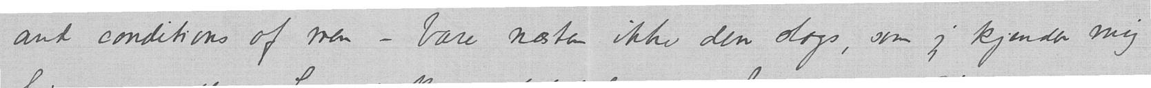and conditions of men – bare næsten ikke den slags, som jeg kjender mig
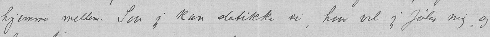hjemme mellem. Saa jeg kan sletikke si, hvor vel jeg føler mig, og
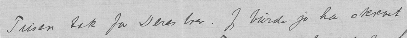Tusen tak for Deres brev. Jeg burde jo ha skrevet
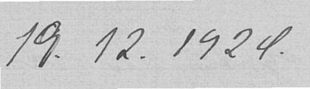19.12.1924.
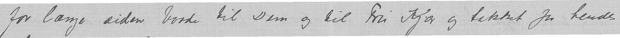for længe siden baade til Dem og til Fru Kjær og takket for hendes
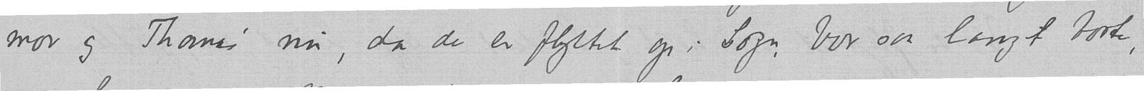mor og Thomas nu, da de er flyttet op i Sogn, bor saa langt borte,
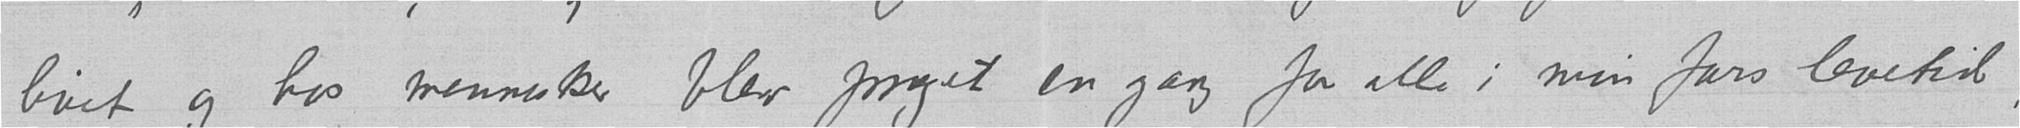livet og hos mennesker blev præget en gang for alle i min fars levetid,
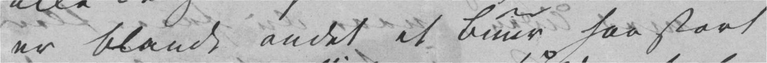er blandt andet et Buur saa stort
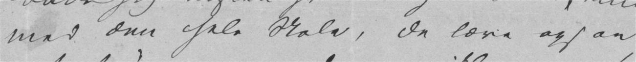med den hele Skole, de lære ogsaa
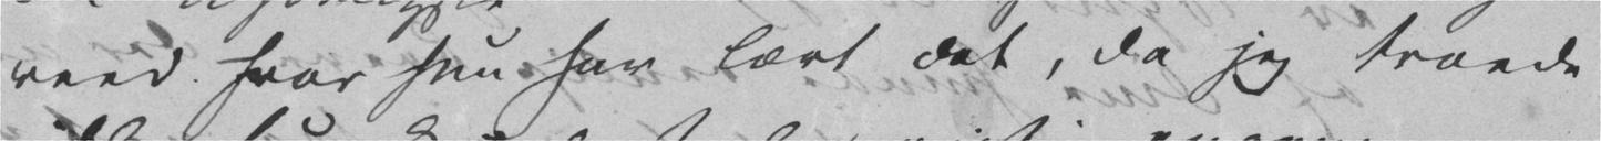veed hvor hun har lært det, da jeg troede
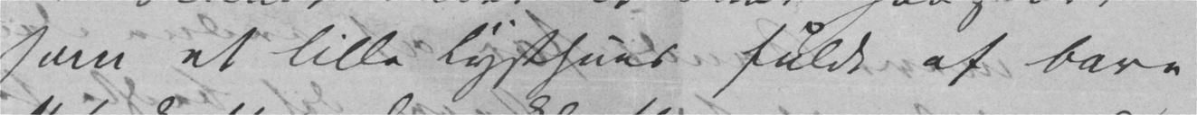som et lille Lysthuus fuldt af bare
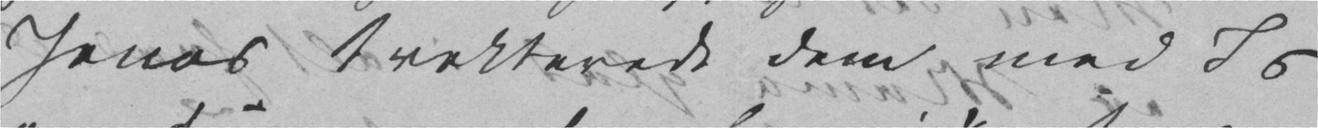Jonas trakterede dem med Is
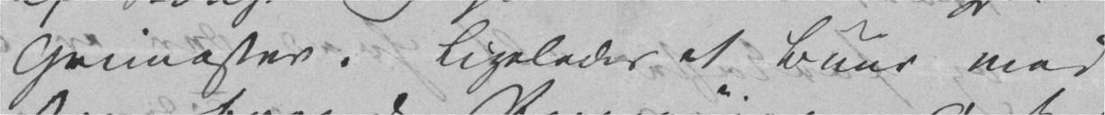Grimasser. Ligeledes et Buur med
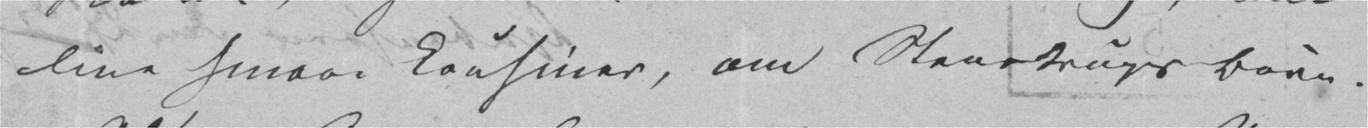dine smaae Cousiner, om Stenstrups Børn.
